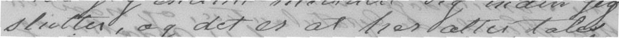slutter, og det er at her atter tales
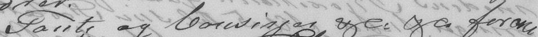Tante og Cousiner ogc. ogc forene
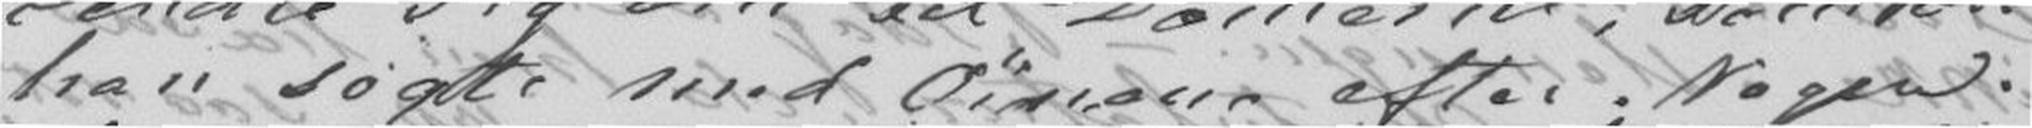han søgte med Øinene efter Nogen.
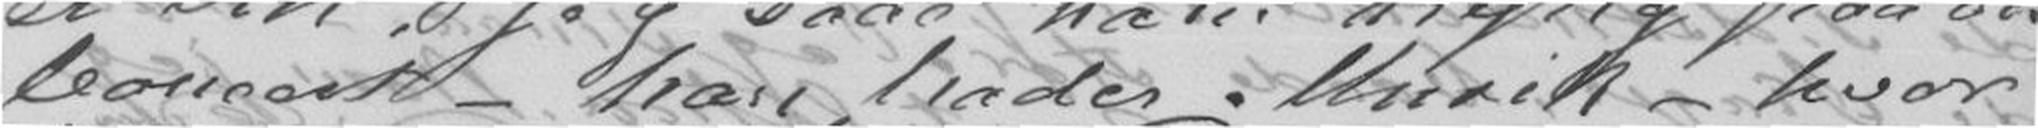Concert - han hader Musik - hvor
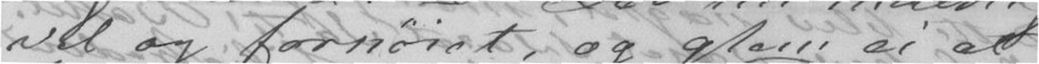vel og fornøiet, og glem ei at
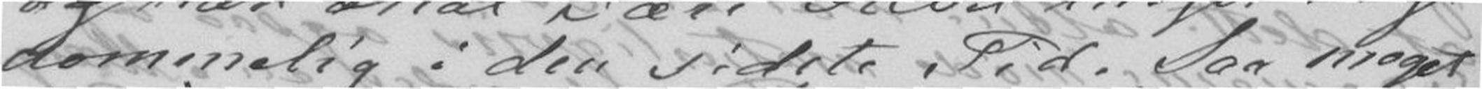dommelig i den sidste Tid. Saa meget
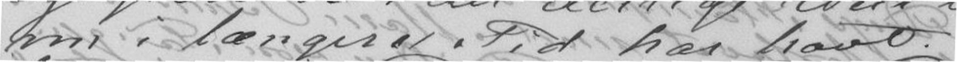nu i længere Tid har havt.
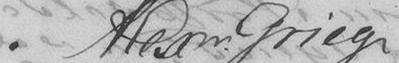Alexr Grieg.
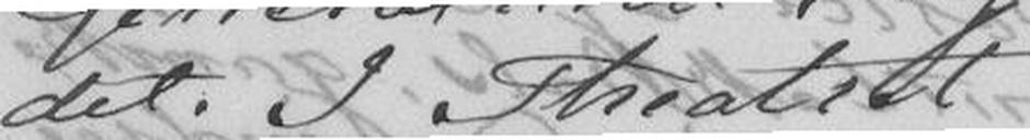det. I Theatret
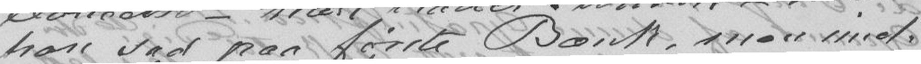han sad paa første Bænk, men imel-
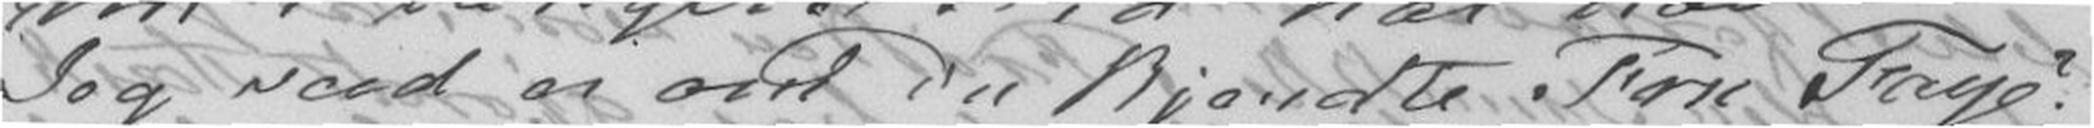Jeg veed ei om Du kjendte Fru Faye?
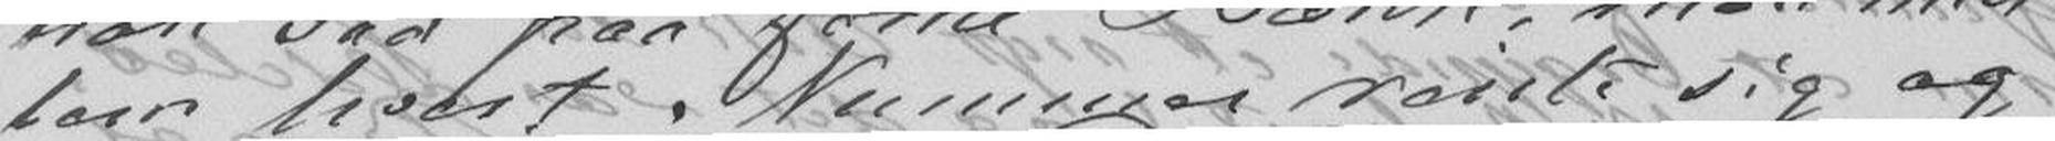lom hvert Nummer reiste sig og
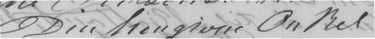Din hengivne Onkel
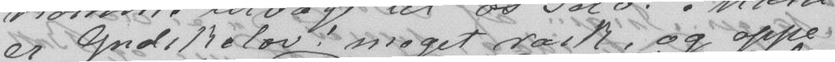er gudskelov! meget rask, og oppe
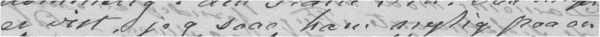er vist, jeg saae ham nylig paa en
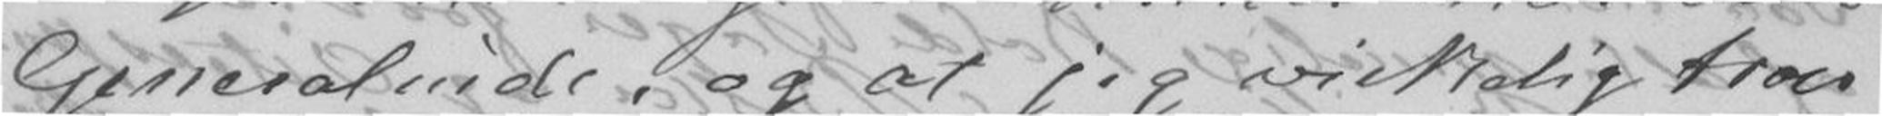Generalinde, og at jeg virkelig troer
